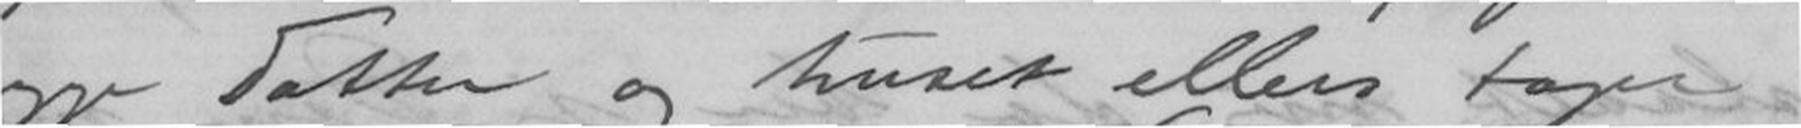syge datter og huset ellers tager
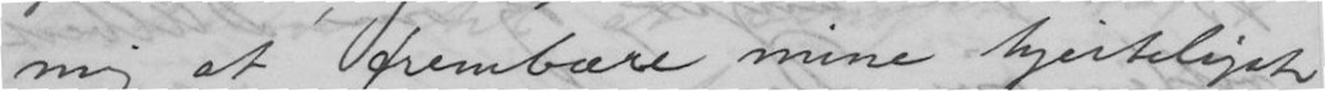mig at frembære mine hjerteligste
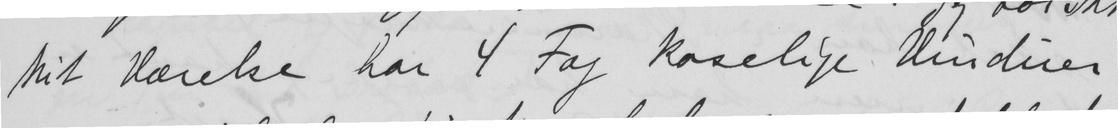Mit Værelse har 4 Fag koselige Vinduer
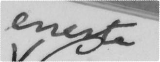eneste
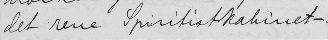det rene Spiritistkabinet -
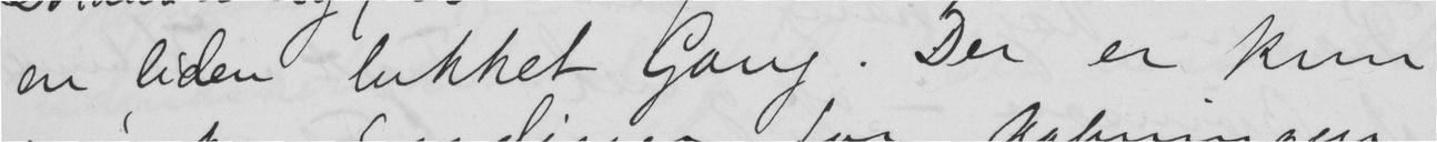en liden lukket Gang. Der er kun
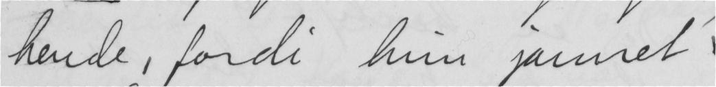hende, fordi hun jamret
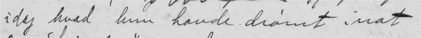idag hvad hun havde drømt inat
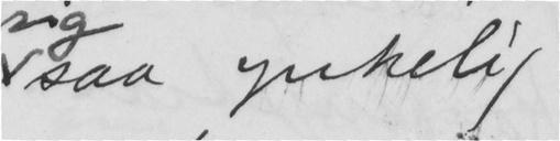saa ynkelig
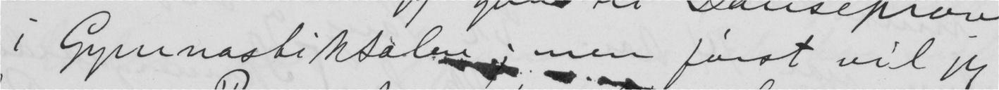i Gymnastiksalen; men først vil jeg
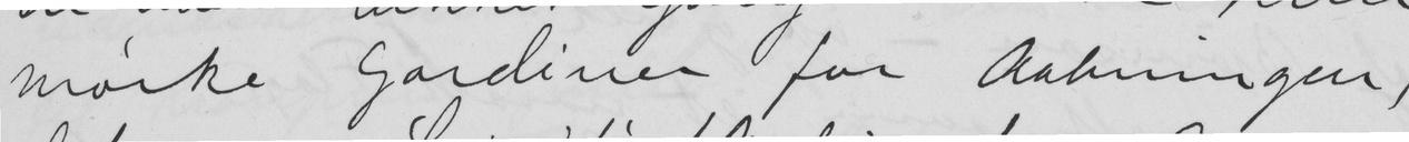mørke Gardiner for Aabningen,
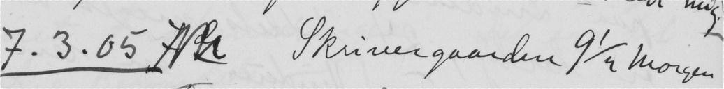7.3.05  Skrivergaarden 9 1/2 Morgen
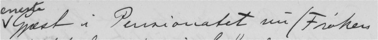Gjæst i Pensionatet nu (Frøken
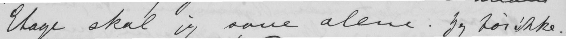Etage skal jeg sove alene. Jeg tør ikke.
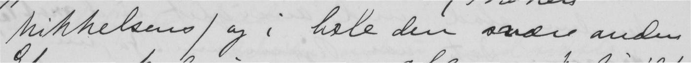Mikkelsens) og i hele den svære anden
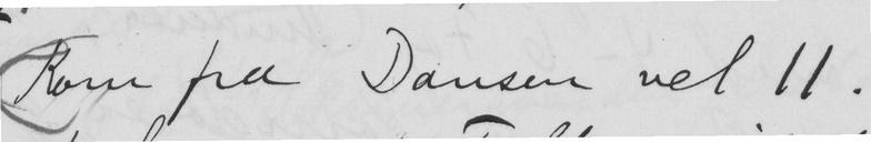Kom fra Dansen vel 11.
